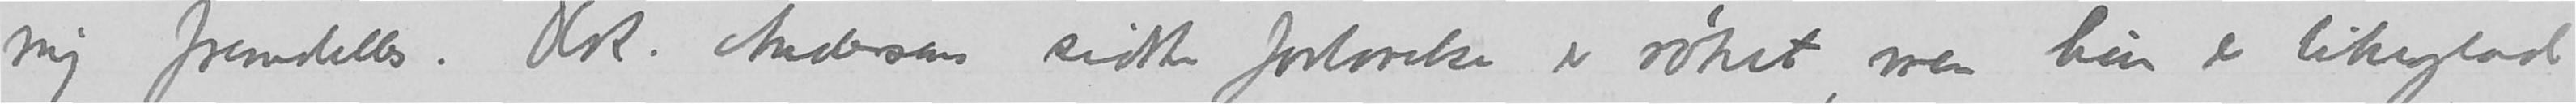mig fremdeles. Frk. Andersens sidste forlovelse er røket, men hun er likeglad
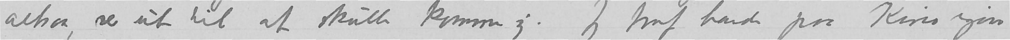altsaa, ser ut til at skulle komme sig. Jeg traf hænde paa Kino igaar
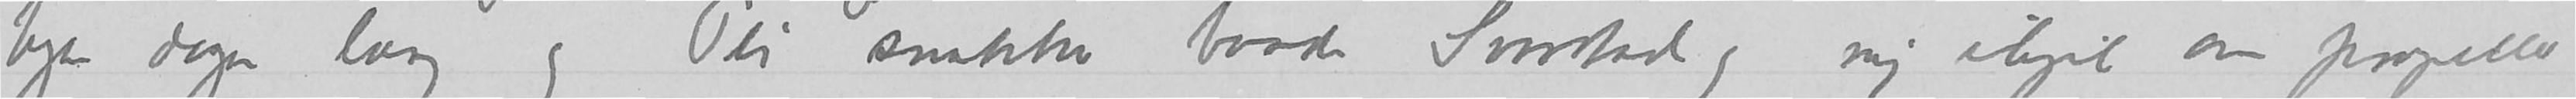byen dagen lang og Pei snakker baade Svarstad og mig ihjel om propeller
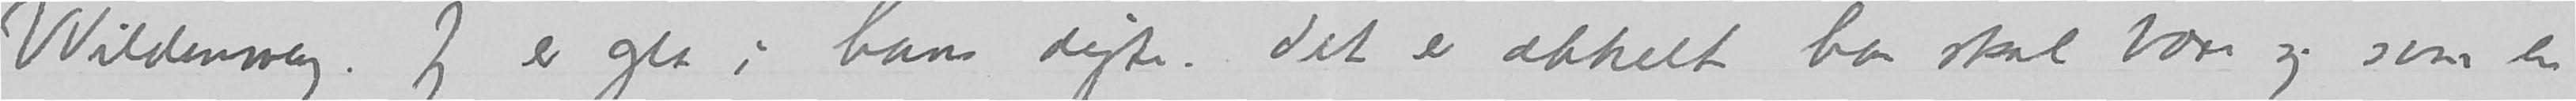Wildenwey.  Jeg er gla i hans digte. det er ækkelt han skal bære sig som en
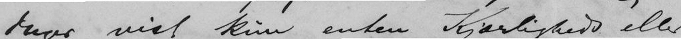duger vist kun enten Kjærligheds eller
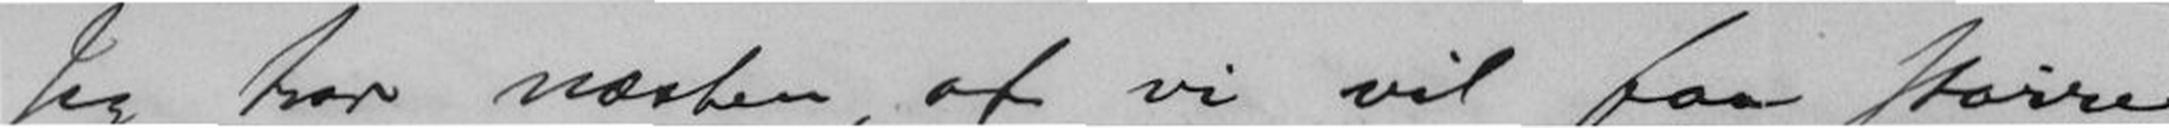Jeg tror næsten, at vi vil faa større
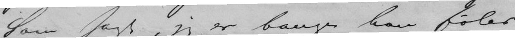Som sagt, jeg er bange han føler
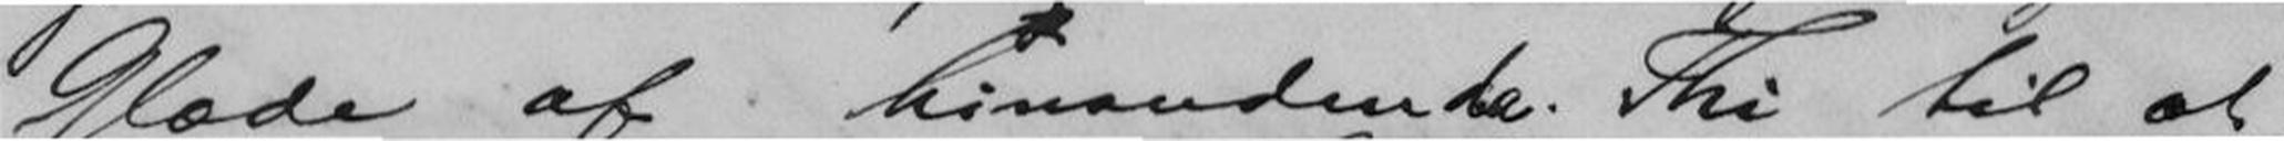Glæde af hinanden da. Thi til at
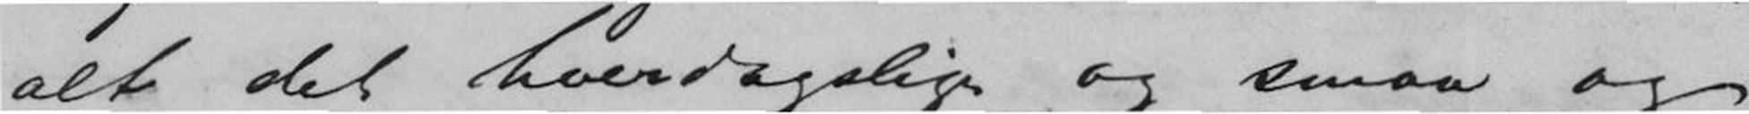alt det hverdagslige og smaa og
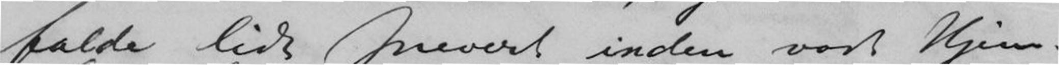falde lidt snevert inden vort Hjem.
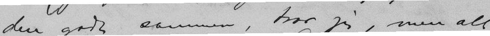den godt sammen, tror jeg, men alt
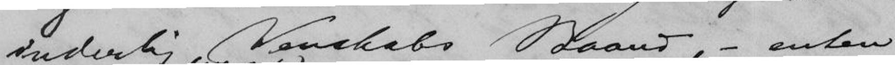inderlig Venskabs Baand, - enten
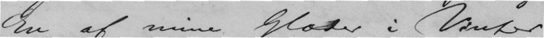En af mine Glæder i Vinter
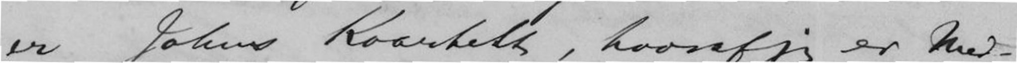er Johns Kvartett, hvoraf jeg er Med-
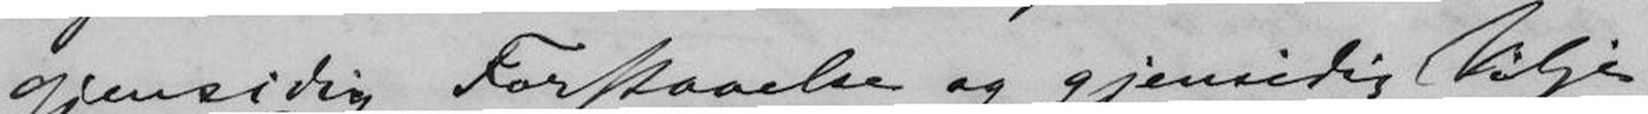gjensidig Forstaaelse og gjensidig Vilje
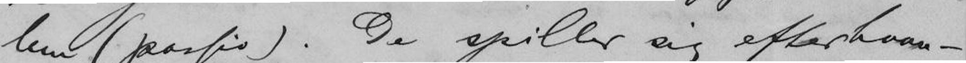lem (passiv). De spiller sig efterhaan-
